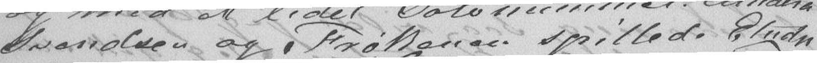Svendsen og Frøkenen spillede Etudn
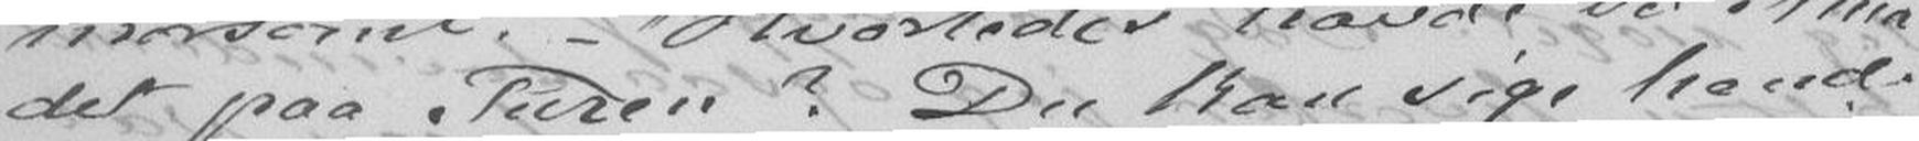det paa Turen? Du kan sige hende
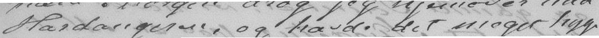Hardangeren, og havde det meget hyg-
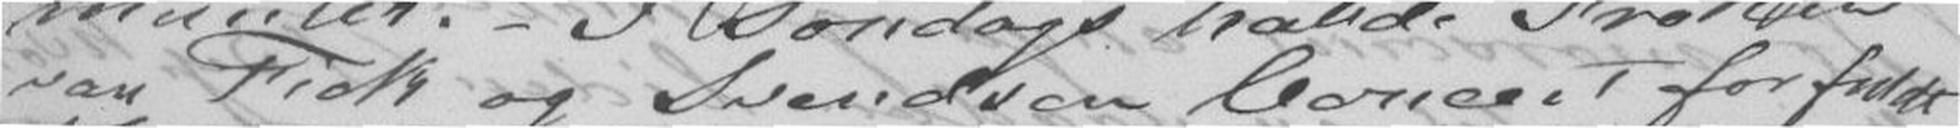van Fick og Svendsen Concert for fuldt
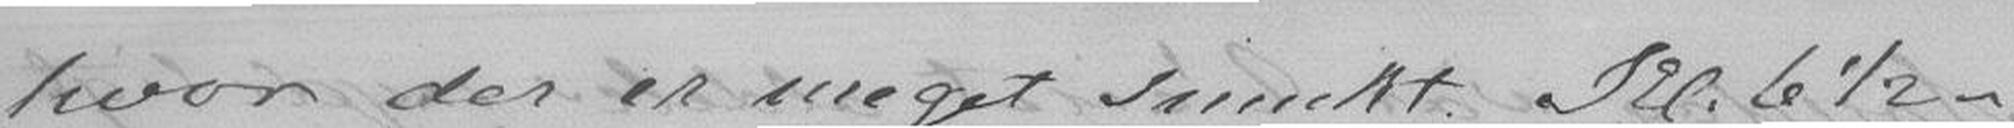hvor der er meget smukt. Kl 6½ -
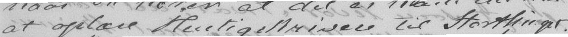at oplære Hurtigskrivelse til Stortingat.
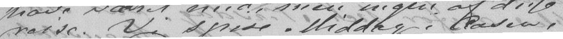reise. Vi spise Middag. Aasee
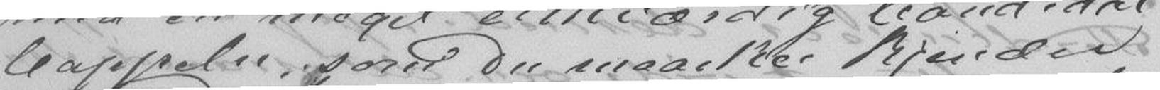Cappeln, som Du maaske kjender
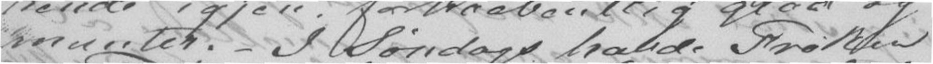munter. - I Søndags havde Frøken
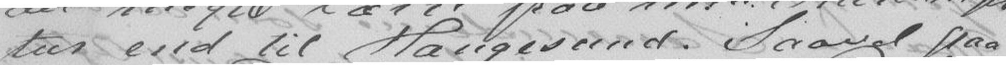tur end til Haugesund. Saavel paa
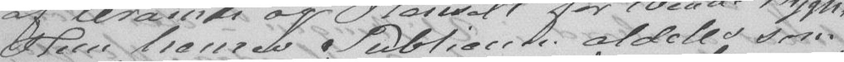Hun henres Publicum aldeles som
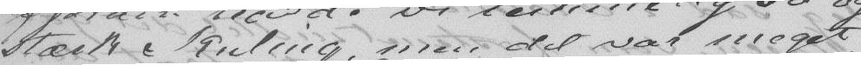stærk Kuling, men det var meget
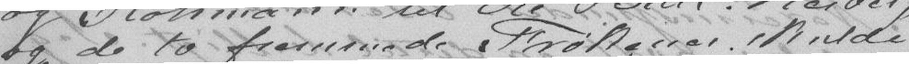og de to fremmede Frøkener skulde
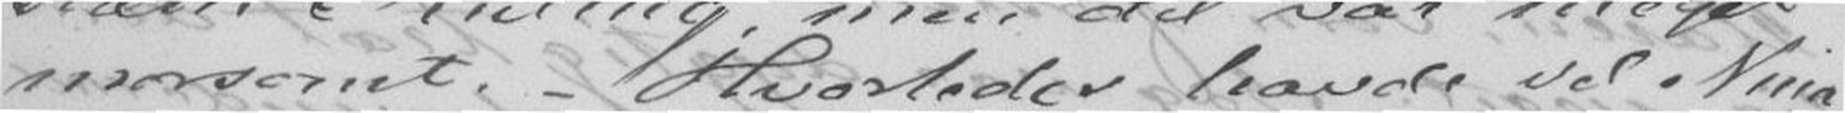morsomt. - Hvorledes havde vel Nina
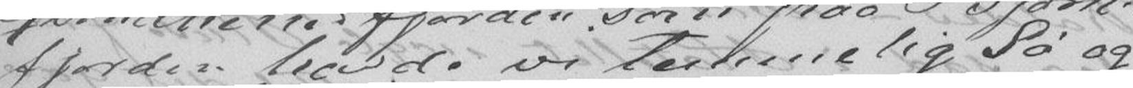fjorden havde vi temmelig Sø og
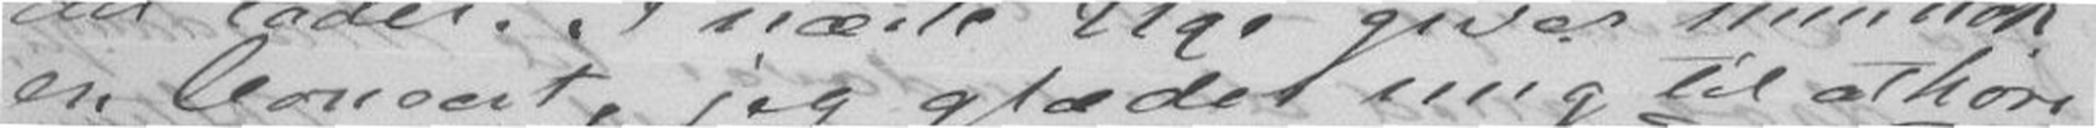en Concert, jeg glæder mig til athøre
